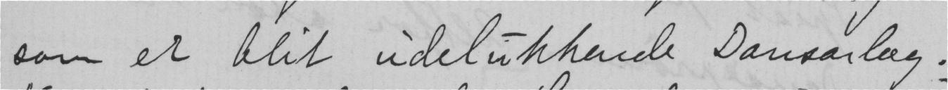som et blit udelukkende Dansarlag.
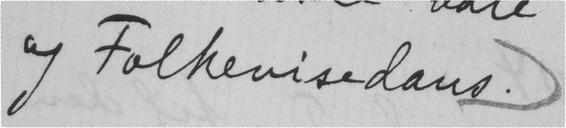og Folkevisedans.
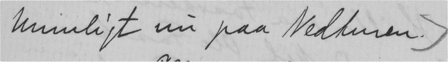umuligt nu paa Nedturen.
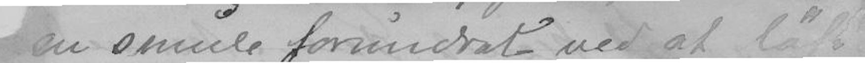en smule forundsat ved at løfte
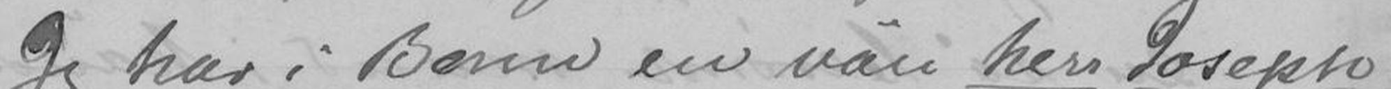Jeg har i Bonn en vän herr Joseph
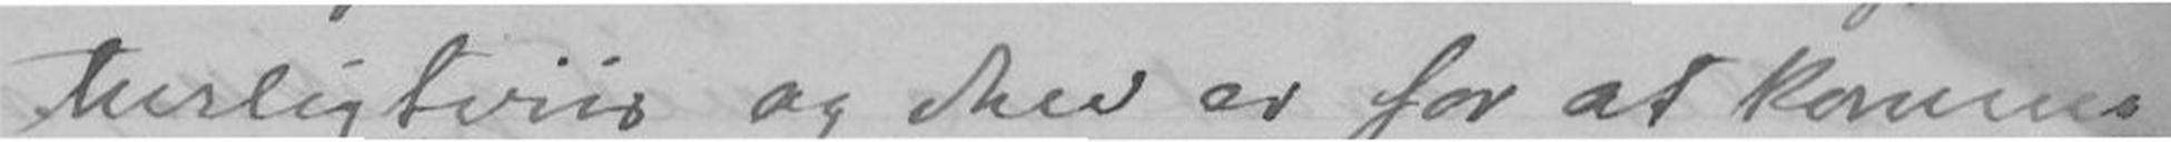turligtvis og den er for at komme
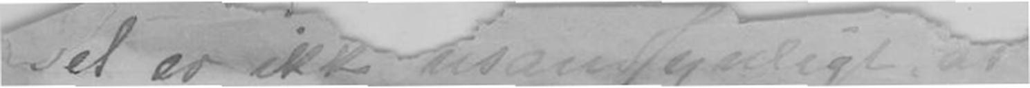Det er ikke usansynligt, at
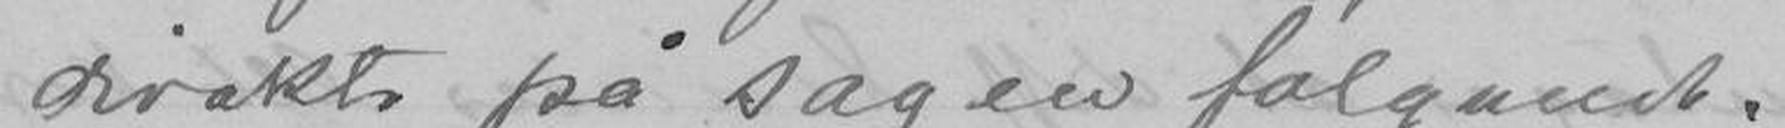direkte på sagen følgende.
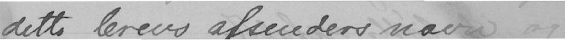dette brevs afsenders navn og
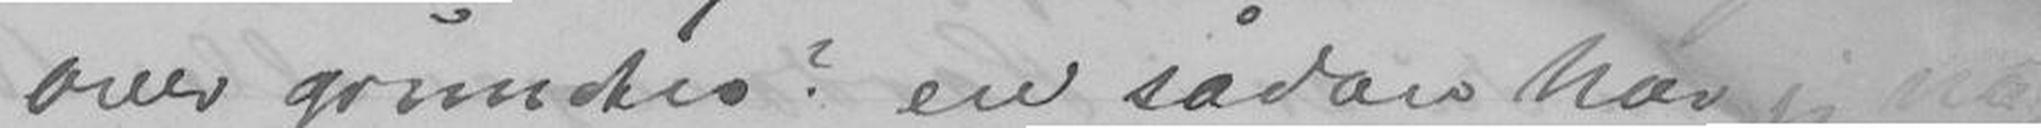over grundes? en sådan har jeg na-
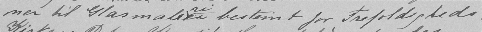ner til Glasmalerier bestemt for Trefoldigheds-
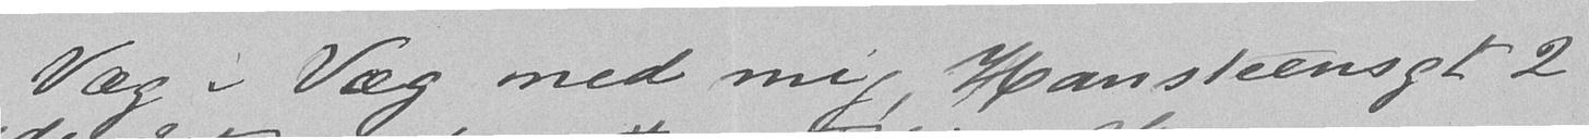Væg i Væg med mig, Hansteensgt. 2
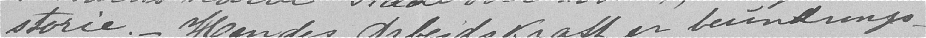storie. Hendes Arbeidskraft er beundrings-
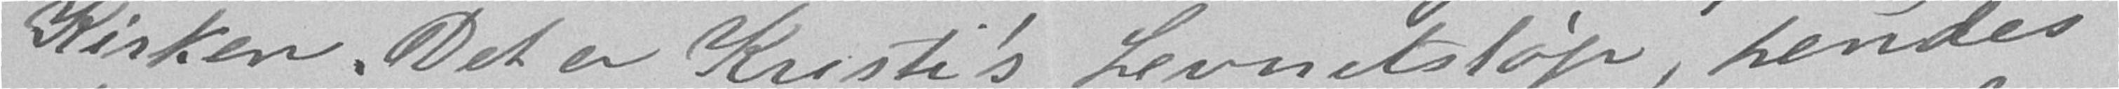Kirken. Det er Kristi's Levnetsløp, hendes
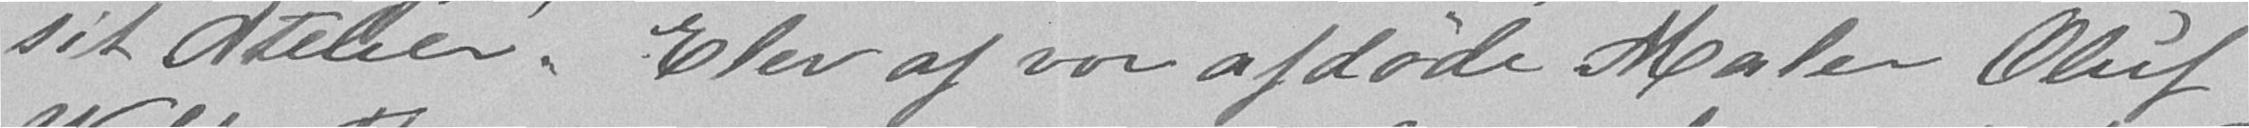sit Atelier. Elev af vor afdøde Maler Oluf
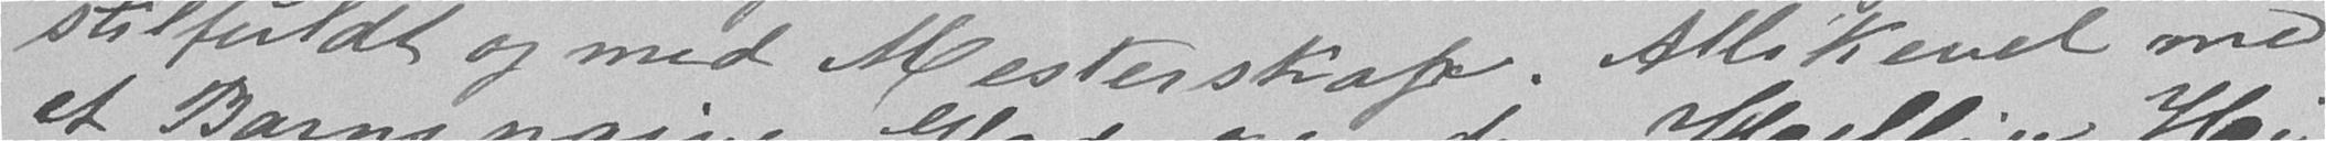stilfuldt og med Mesterskap. Allikevel med
